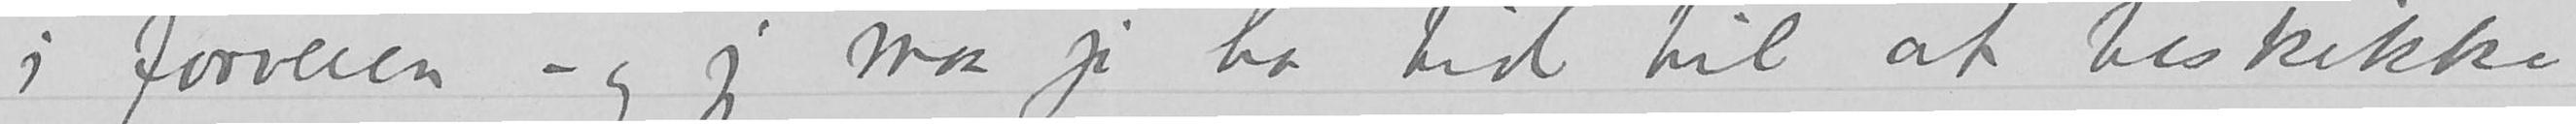i forveien – jeg jeg maa jo ha tid til at beskikke
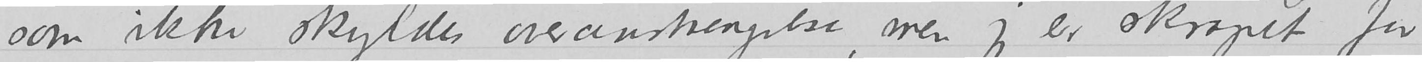som ikke skyldes overanstrengelse, men jeg er skrapet for
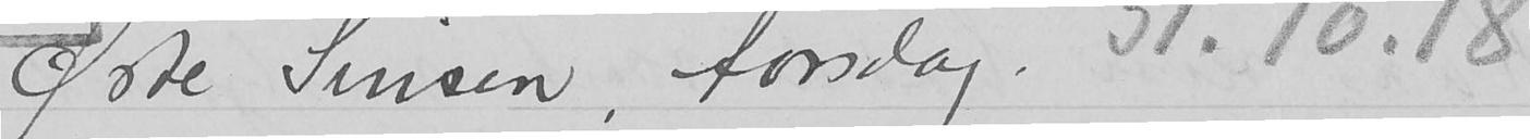Østre Sinsen, torsdag
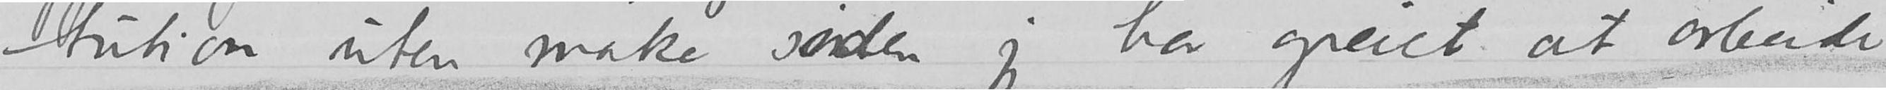tution uten make siden jeg har greiet at arbeide
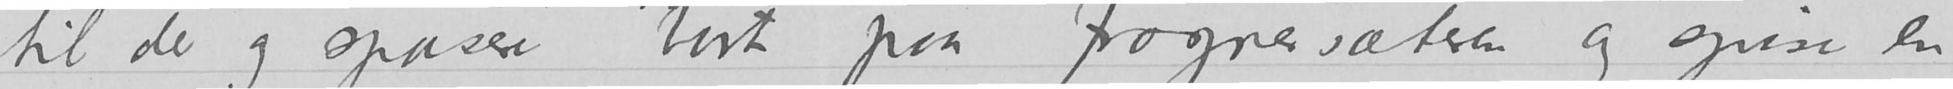til der og spasere bort paa Frognersæteren og spise en
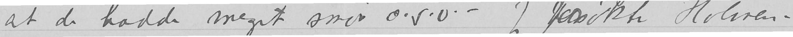at de hadde meget smør o.s.v. – Jeg forsøkte Holmen
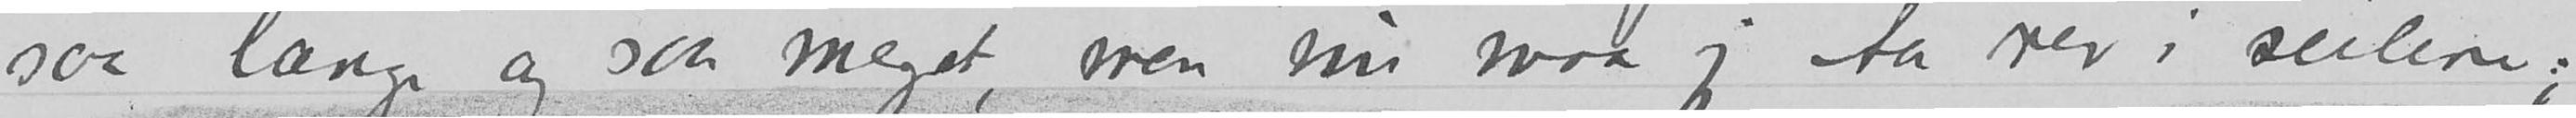saa længe og saa meget, men nu maa jeg ta rev i seilene;
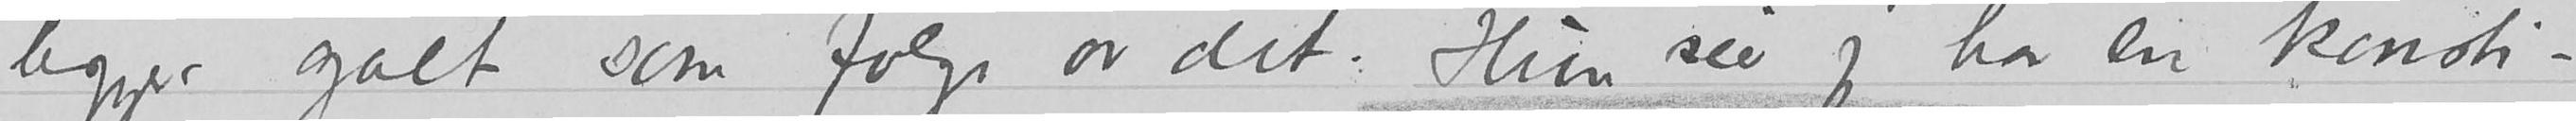ligger galt som følge av det. Hun sier jeg har en konsti-
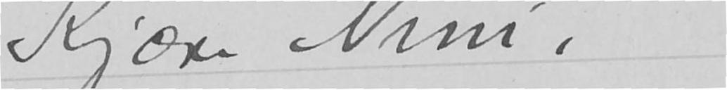Kjære Nini,
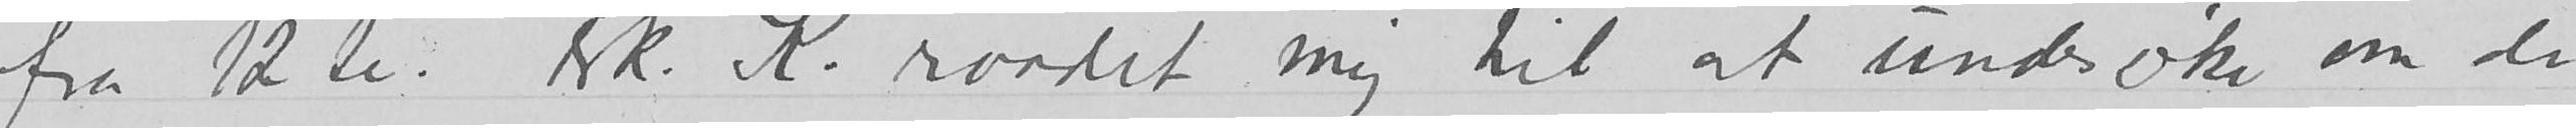fra 12te. Frk. K. raadet mig til at undersøke om de
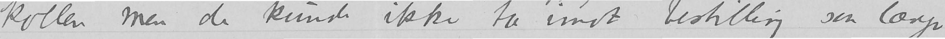kollen men de kunde ikke ta imot bestilling saa længe
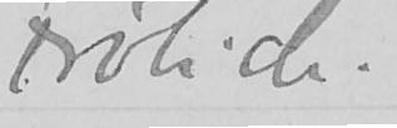Frølich.
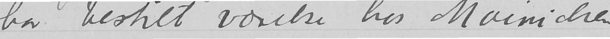Jeg har bestilt værelse hos Møinichen
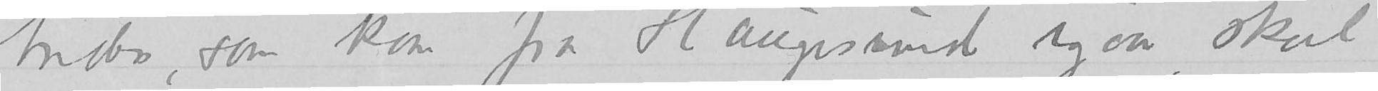Anders, som kom fra Haugesund igaar, skal
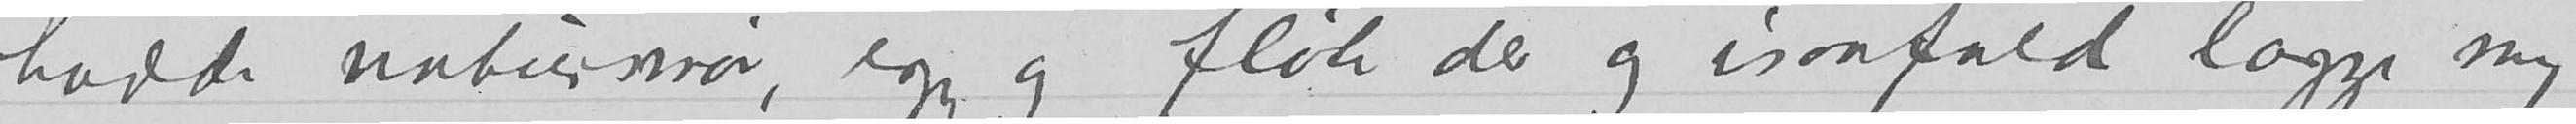hadde nabusmør, egg og fløte der og isaafald lægge mig
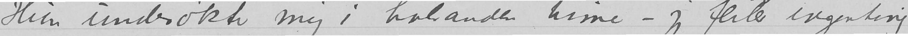Hun undersøkte mig i halvanden time – jeg feiler ingenting
